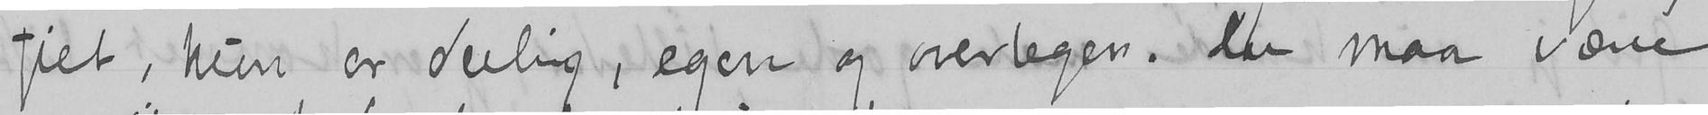fiet, hun er deilig, egen og overlegen. Du maa være
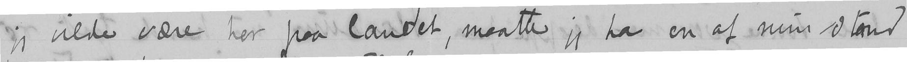jeg vilde være her paa landet, maatte jeg ha en af min stand
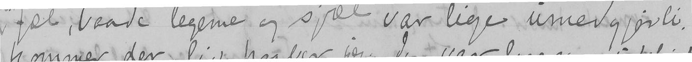fæl, baade legeme og sjæl var lige umedgjørli-
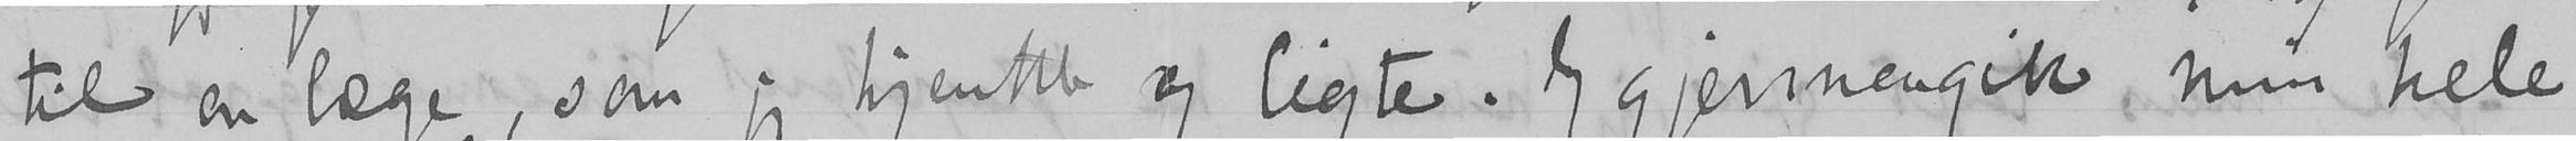til en læge, som jeg kjentte og ligte. Jeg gjennemgik min hele
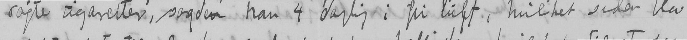røgte cigaretter, sagden han 4 daglig i fri luft, hvilket siden blev
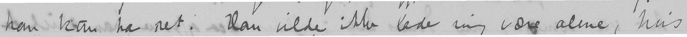han kan ha ret. Han vilde ikke lade mig være alene, hvis
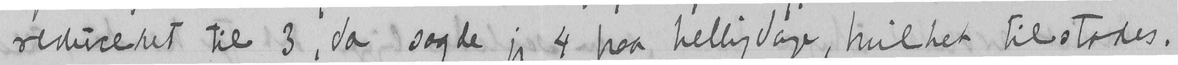reduceret til 3, da sagde jeg 4 paa helligdage, hvilket tilstedes.
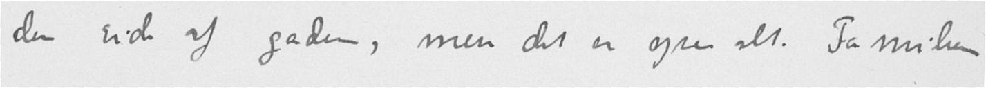den side af gaden, men det er ogsaa alt. Familien
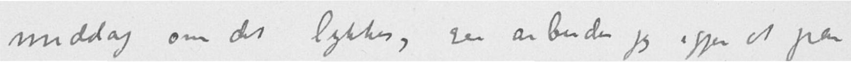middag om det lykkes, saa arbeider jeg igjen et par
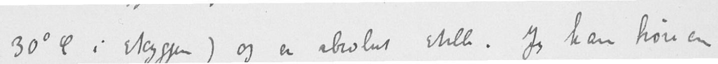30⁰ C i skyggen) og er absolut stille. Jeg kan høre en
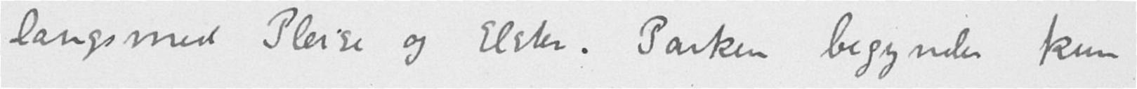langsmed Pleise og Elster. Parken begynder kun
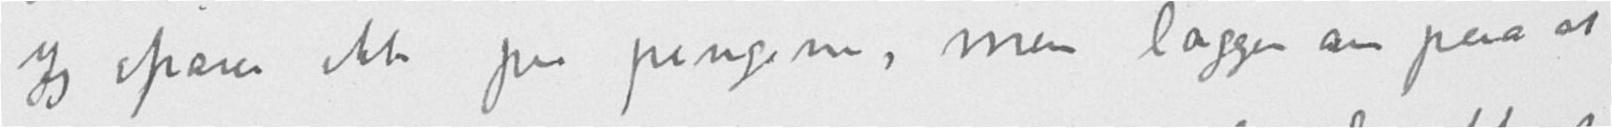Jeg sparer ikke paa pengene, men lægger an paa at
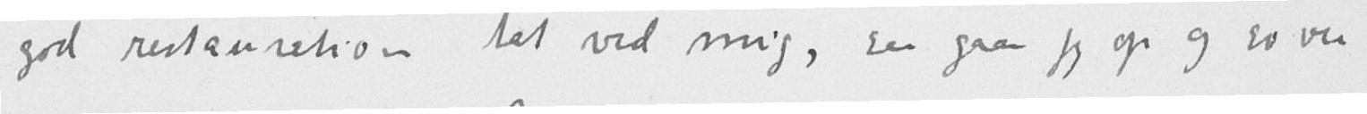god restauration tet ved mig, saa gaar jeg op og sover
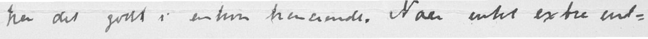have det godt i enhver henseende. Naar intet extra ind-
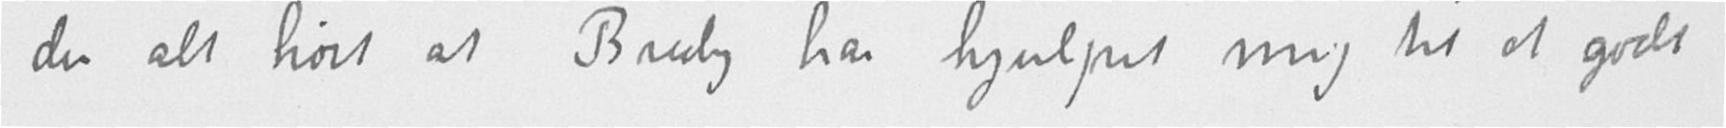du alt hørt at Bredig har hjulpet mig til et godt
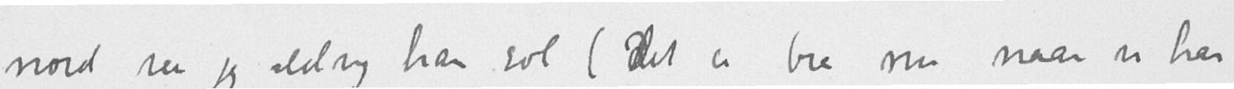nord saa jeg aldrig har sol (det er bra nu naar vi har
